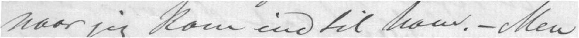naar jeg kom ind til ham. - Men
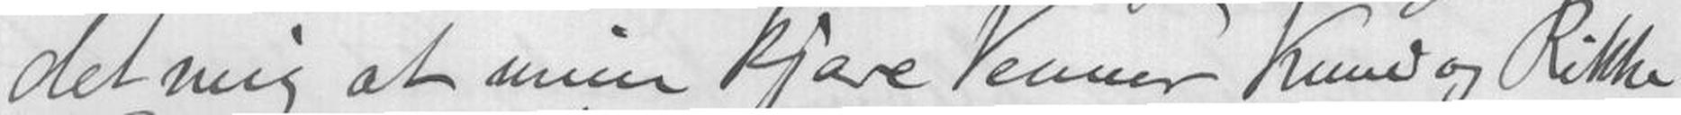det mig at mine kjære Venner Knud og Rikke
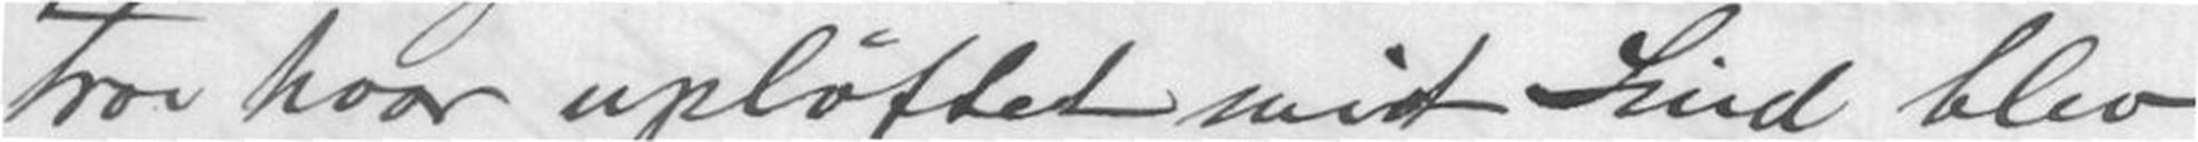troe hvor upløftet mit Sind blev
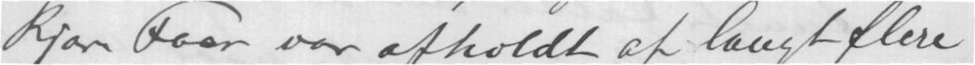kjære Faer var afholdt af langt flere
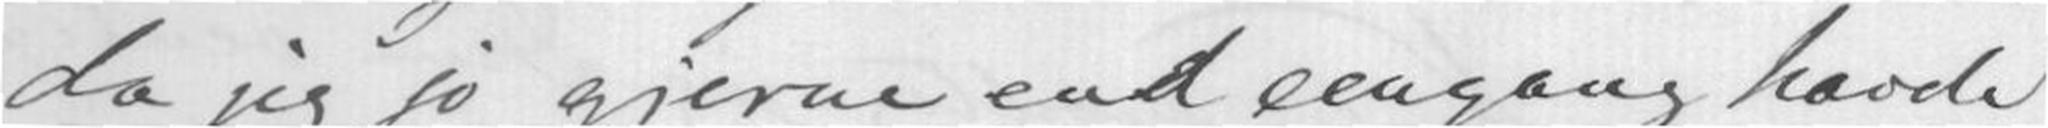da jeg jo gjerne end eengang havde
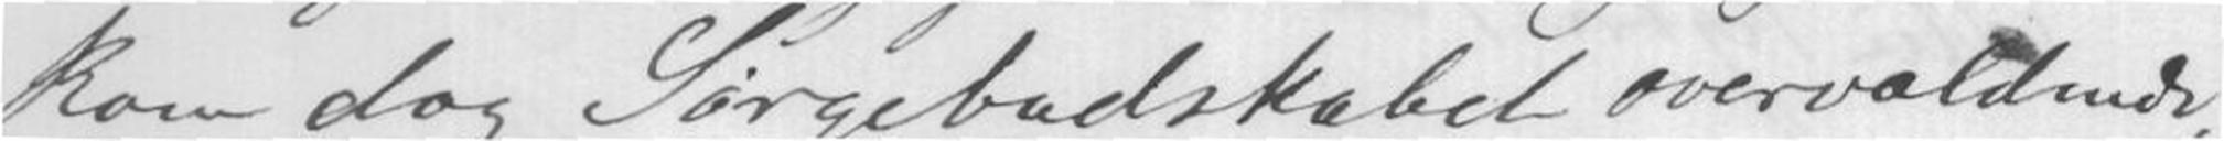kom dog Sørgebudskabet overvældende,
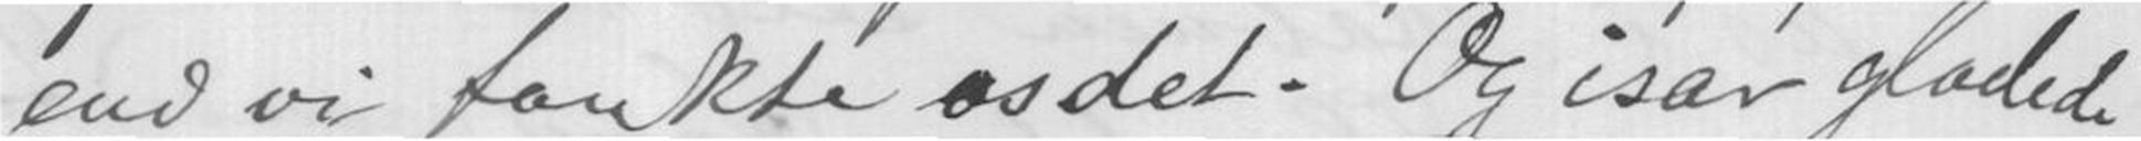end vi tænkte os det. Og især glædede
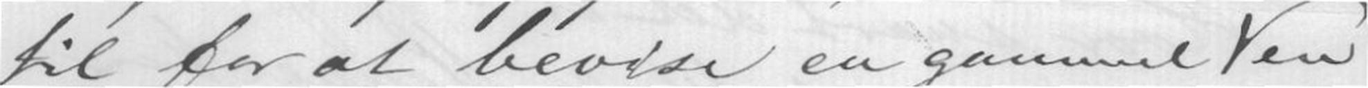til for at bevise en gammel Ven
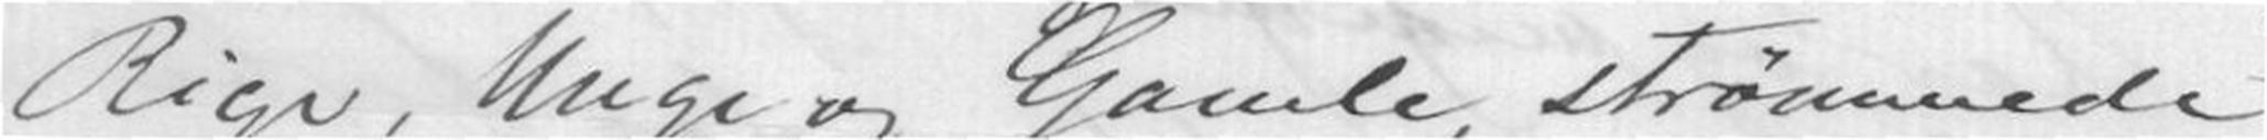alle Veninder og Venner, strømmede
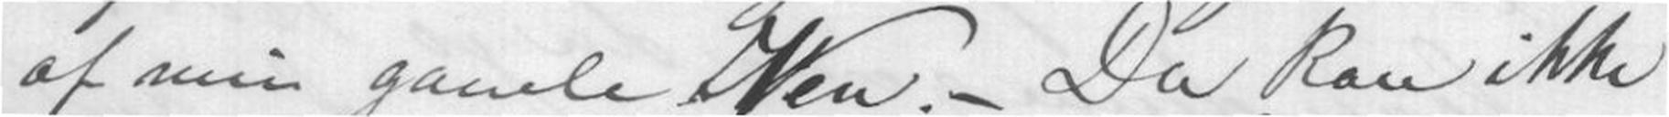af min gamle Ven. - Du kan ikke
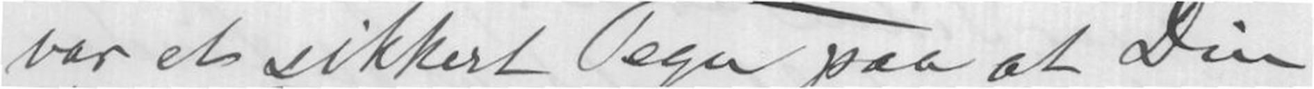var et sikkert Tegn paa at Din
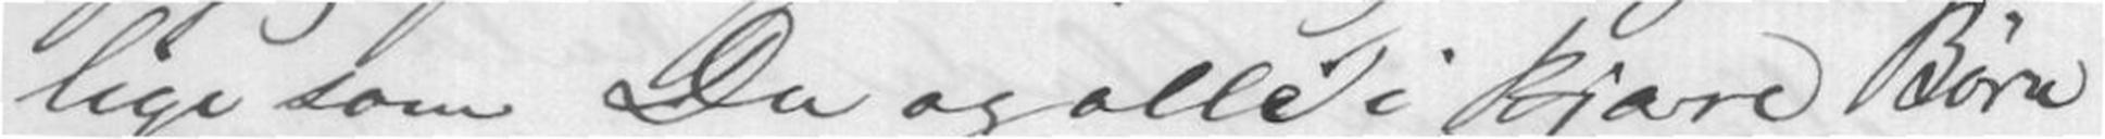lige som Du og alle i kjære Børn
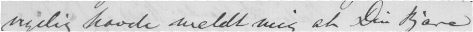visslig havde meldt mig at Din kjære
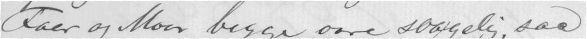Faer og Moer begge vare svagelig, saa
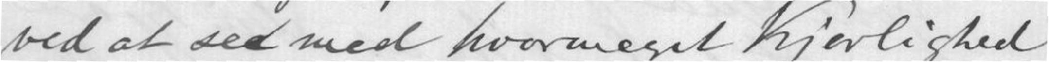ved at see med hvormeget kjærlighed
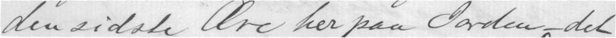den sidste Ære her paa Jorden det
